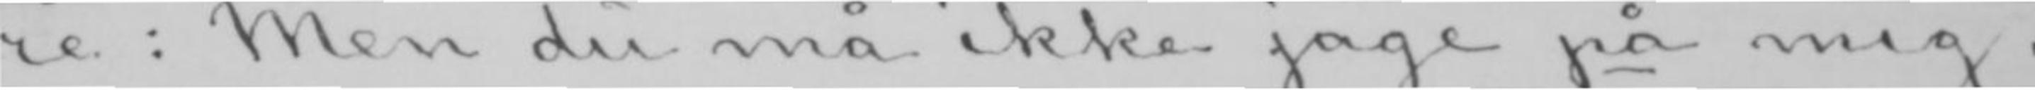re: Men du må ikke jage på mig.
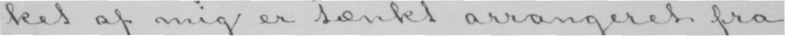ket af mig er tænkt arrangeret fra
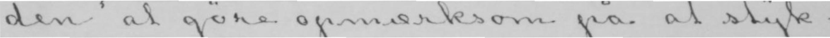den» at gøre opmærksom på at styk-
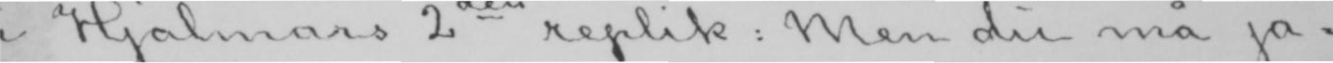i Hjalmars 2den replik: Men du må ja-
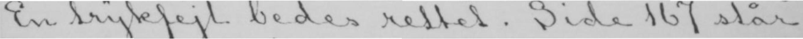En trykfejl bedes rettet. Side 167 står
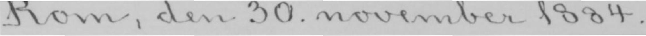Rom, den 30. november 1884.
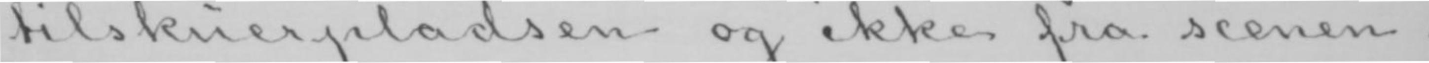tilskuerpladsen og ikke fra scenen.
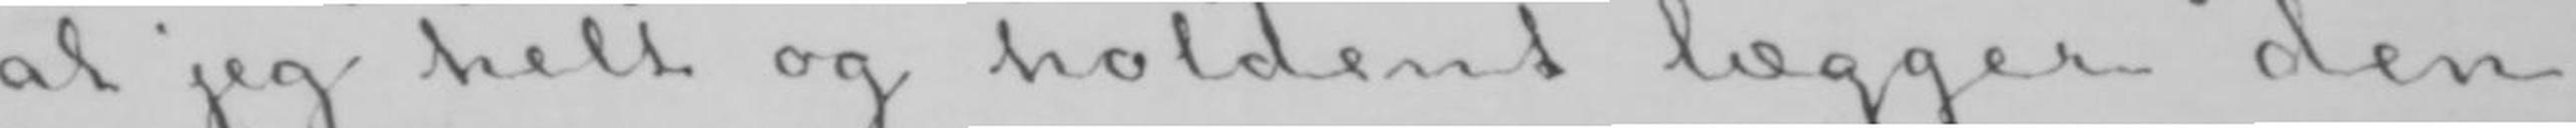at jeg helt og holdent lægger den
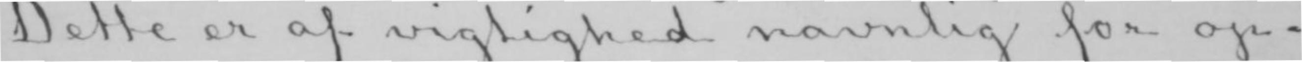Dette er af vigtighed navnlig for op-
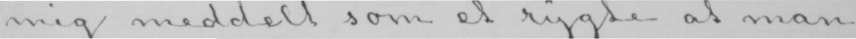mig meddelt som et rygte at man
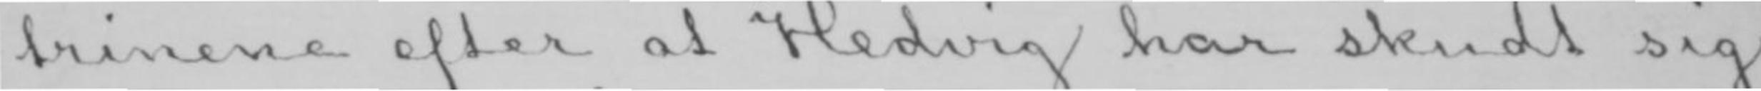trinene efter at Hedvig har skudt sig.
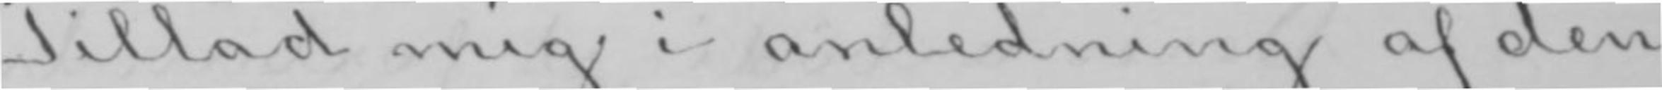Tillad mig i anledning af den
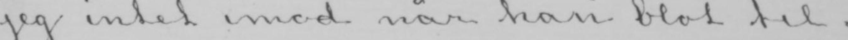jeg intet imod når han blot til-
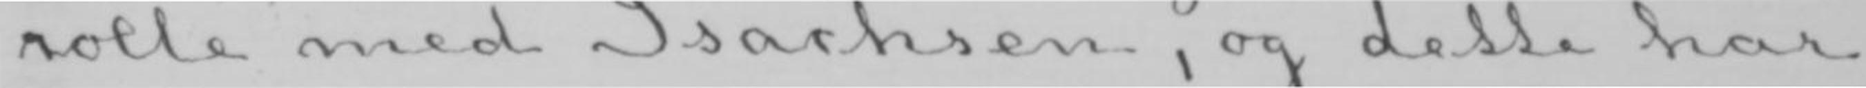rolle med Isachsen, og dette har
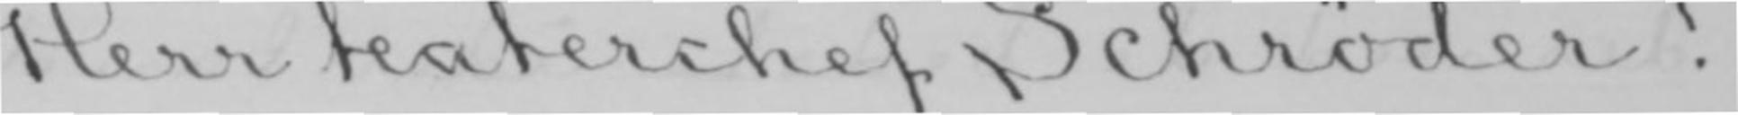Herr teaterchef Schrøder!
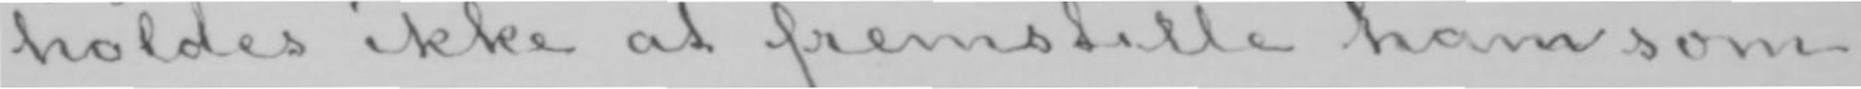holdes ikke at fremstille ham som
